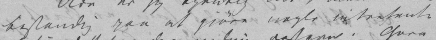bestandig paa at gjøre nogle intresante
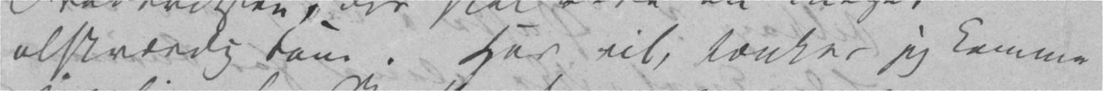elskværdig Kone. Her vil, tænker jeg komme
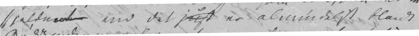sjeldent end det just er almindeligt blandt
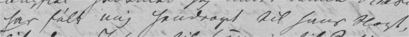har følt mig hendraget til hans Slægt,
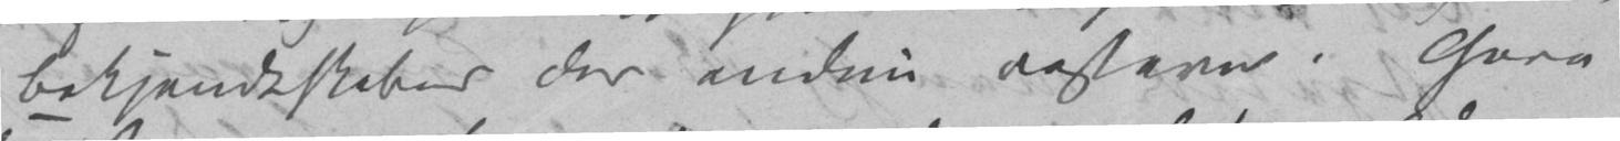Bekjendtskaber der endnu restere. Thora
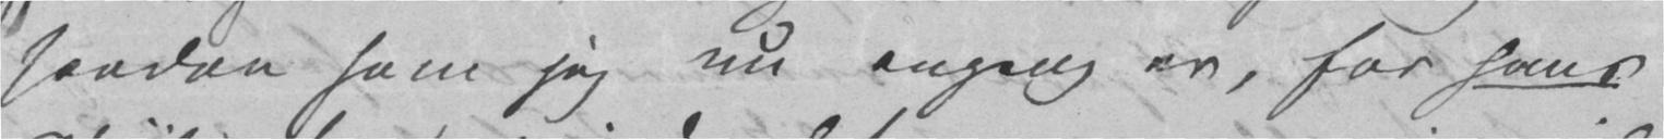saadan som jeg nu engang er, for hans
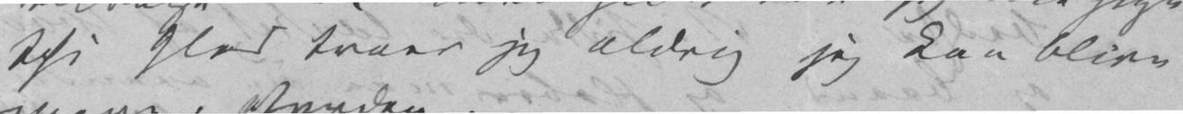thi glad troer jeg aldrig jeg kan blive
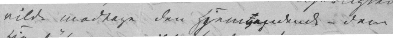vilde modtage den Hjemvendende – den
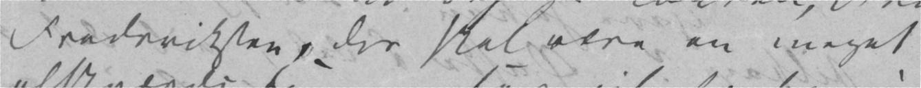Frederiksen, der skal være en meget
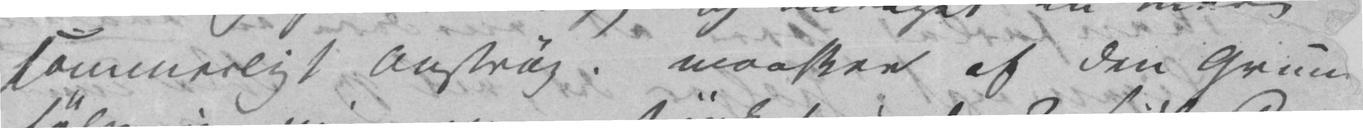sommerligt Anstrøg. maaskee af den Grun
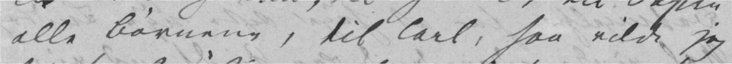alle Børnene, til Carl, saa vilde jeg
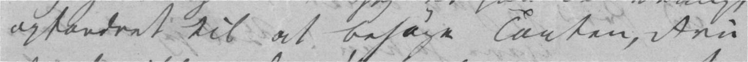opfordret til at besøge Tanten, Fru
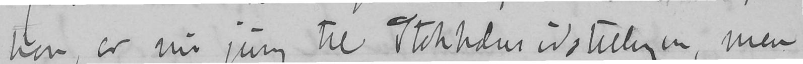tion, er nu jury til Stokholmudstillingen, men
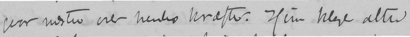gaar næsten over hendes kræfter. Hun klage altid
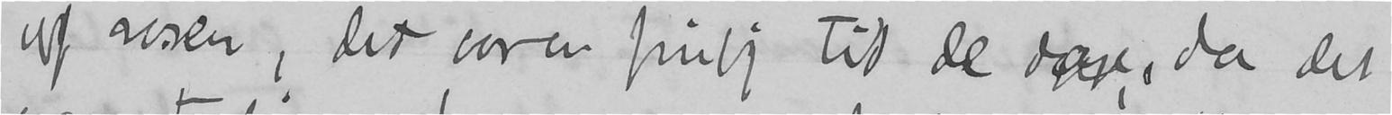gs avisen, det var en pinlig tid de dage, da det
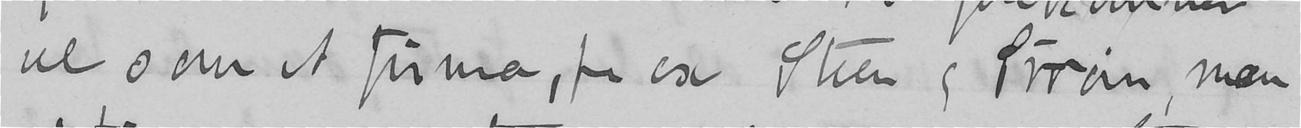vel som et firma, fr ex Steen og Strøm, man
